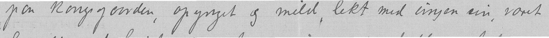paa kongsgaarden, opynget og mild, lekt med ungen sin, været
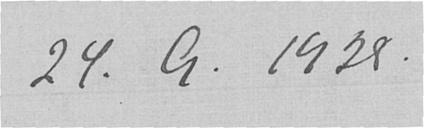24.9. 1928.
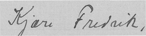Kjære Fredrik,
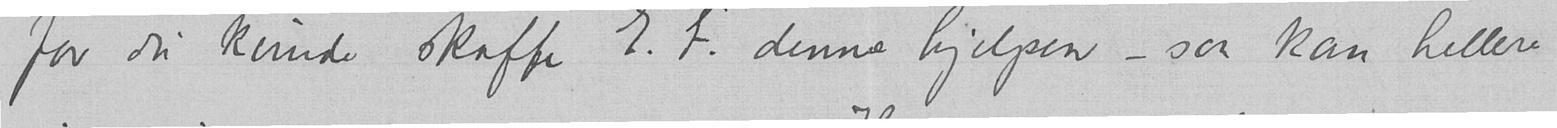for du kunde skaffe E.F. denne hjelpen – saa kan hellere
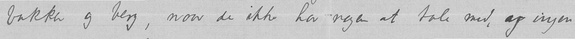bakker og berg, naar de ikke har nogen at tale med, og ingen
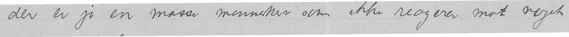der er jo en masse menneske som ikke reagerer mot noget
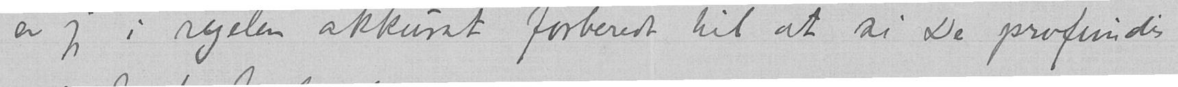er jeg i regelen akkurat forberedt til at si De profundis
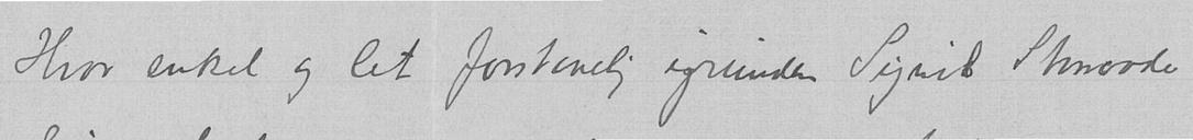Hvor enkel og let forstaaelig igrunden Sigrid Storaade
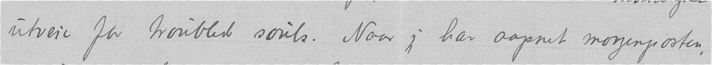utveie for troubled souls. Naar jeg har aapnet morgenposten,
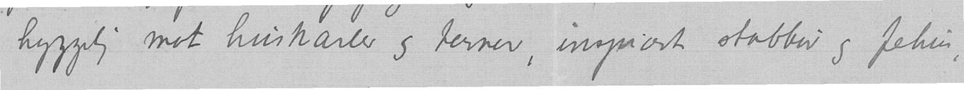hyggelig mot huskarler og terner, inspicert stabbur og fehus,
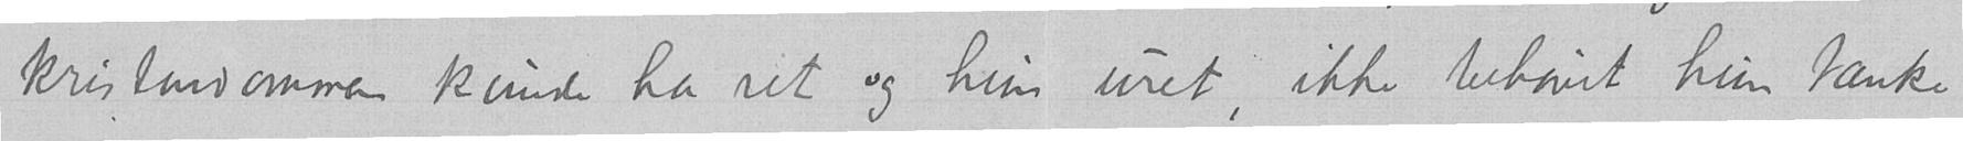kristendommen kunde ha ret og hun uret, ikke behøvet hun tænke
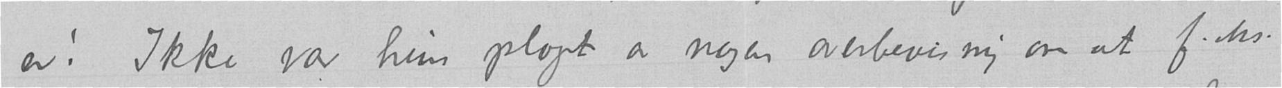er! Ikke var hun plaget av nogen overbevismig om at f.eks.
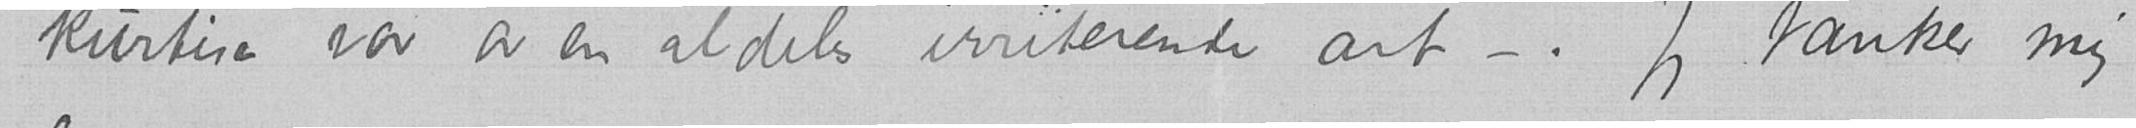kurtise var av en aldeles irriterende art –. Jeg tænker mig
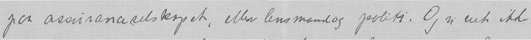paa assuranceselskapet, eller lensmand og politi. Og vi vet ikke
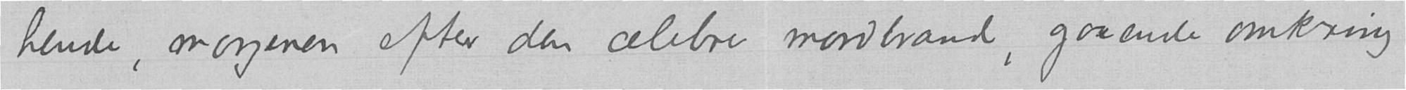hende, morgenen efter den celebre mordbrand, gaaende omkring
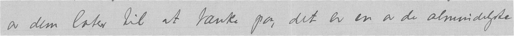av dem later til at tænke paa, det er en av de almindeligste
